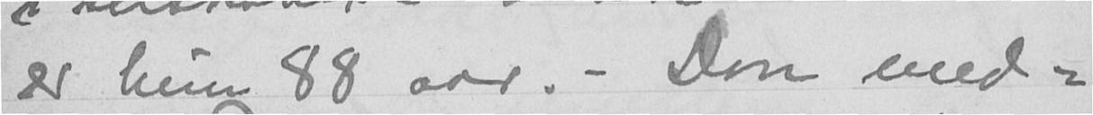er hun 88 aar. - Don med-
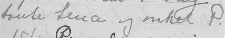tante Lena og onkel P.
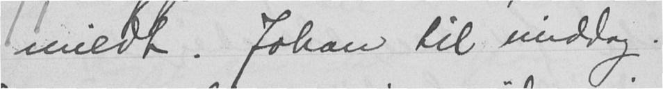mildt. Johan til middag.
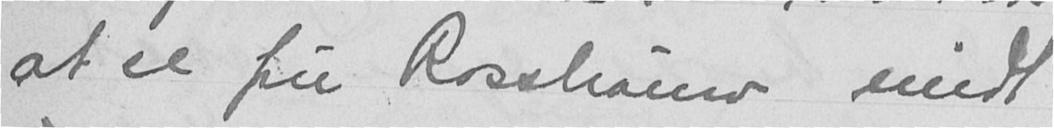at se fru Rosshauw midt
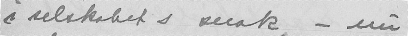i selskabets snak - nu
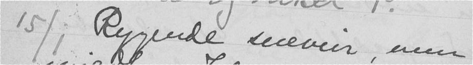15/1 Rygende sneveir, men
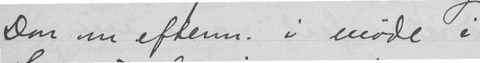Don om efterm. i møde i
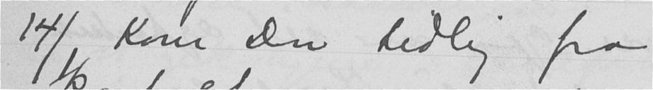14/1 Kom Don tidlig fra
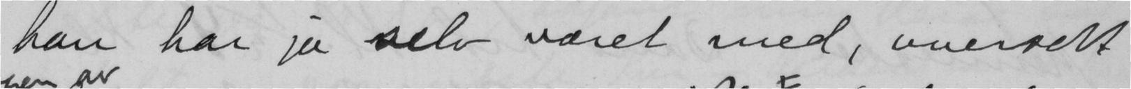han har jo selv været med, oversett
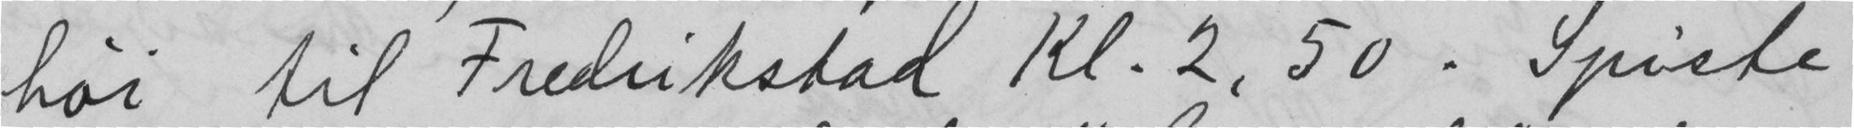høi til Fredrikstad Kl. 2,50. Spiste
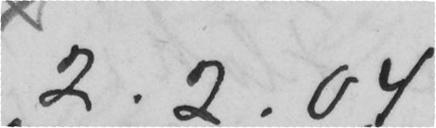2.2.04
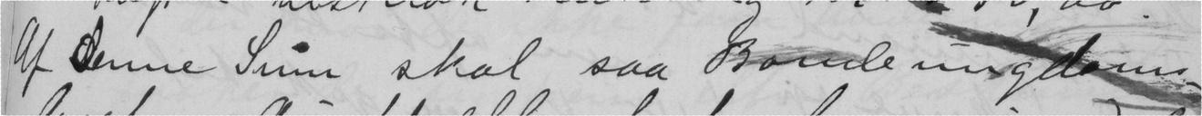Af denne Sum skal saa Bonde ungdoms-
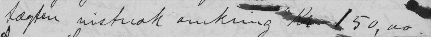tægten vinstnok omkring Kr 150,00.
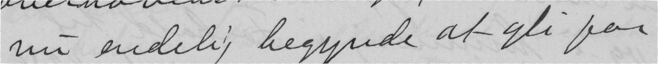nu endelig begynde at gli for
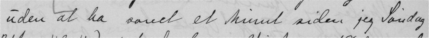uden at ha sovet et Minut siden jeg Søndag
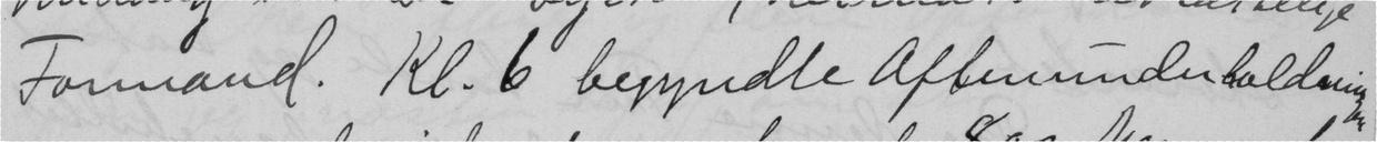Formand. Kl. 6 begyndte aftenunderholdnigen
